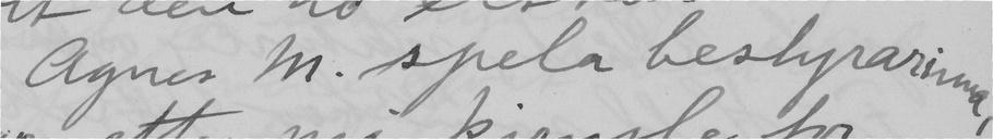Agnes M. spela bestyrarinna,
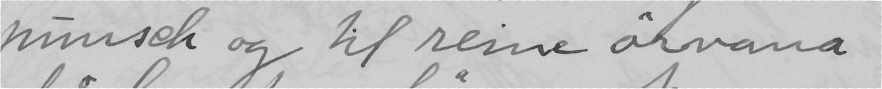punsch og til reine örvana
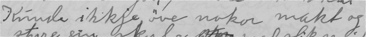Kunde ikkje öve nokon makt og
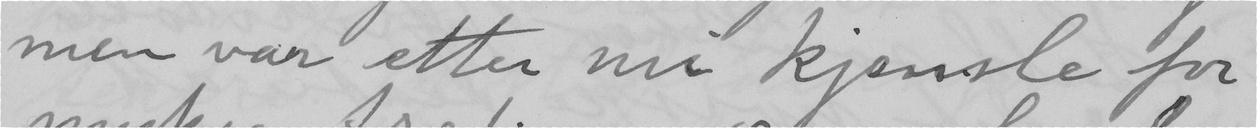men var etter mi kjensle for
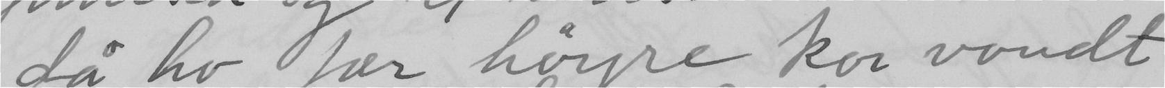då ho fær höyre kor vondt
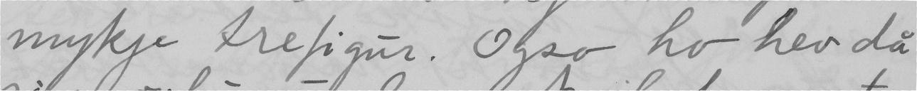mykje trefigur. Ogso ho hev då
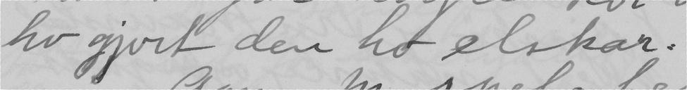ho gjort den ho elskar.
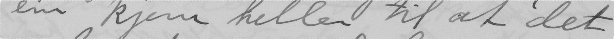ein kjem heller til at det
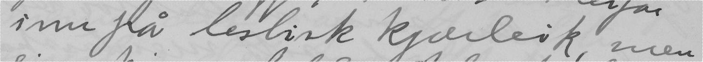inn på lesbisk kjærleik, men
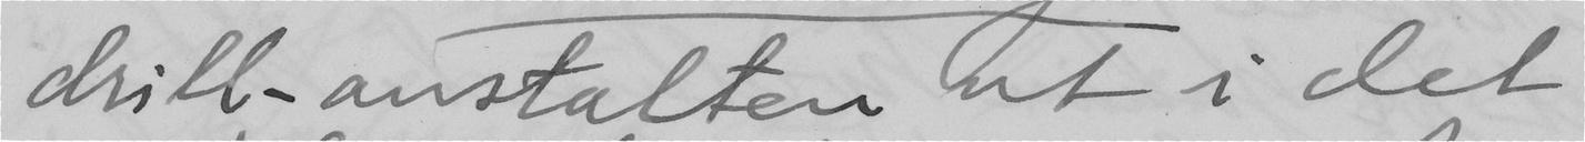drill-anstalten ut i det
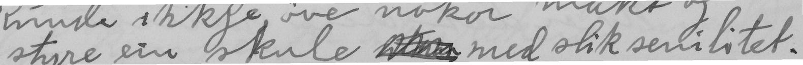styre ein skule med slik senilitet.
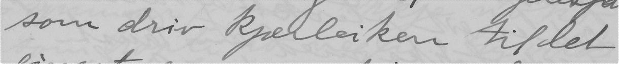som driv kjærleiken til det
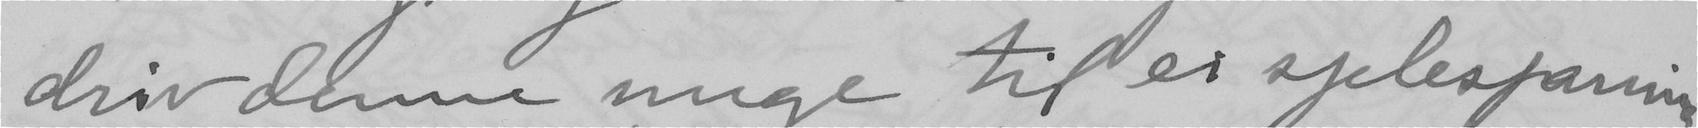driv denne unge til ei sjelespaning
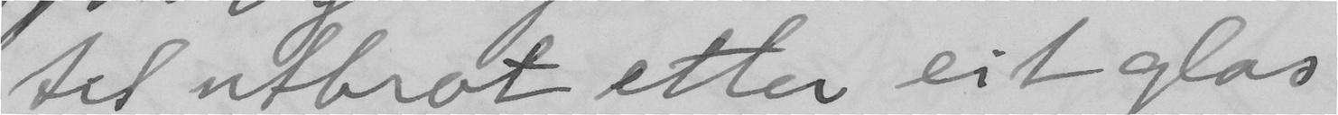til utbrot etter eit glas
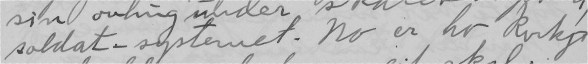soldat-systemet. No er ho korkje
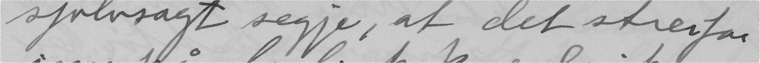sjölvsagt segje, at det streifar
